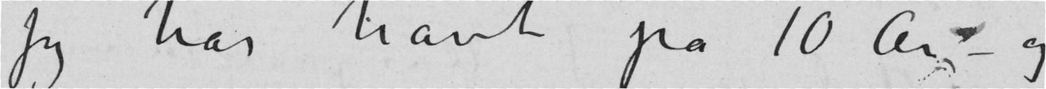jeg har havt på 10 År – og
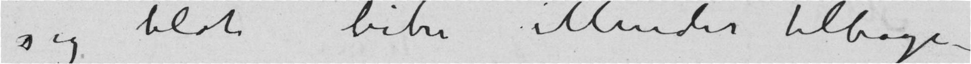sig blot bitre Minder tilbage –
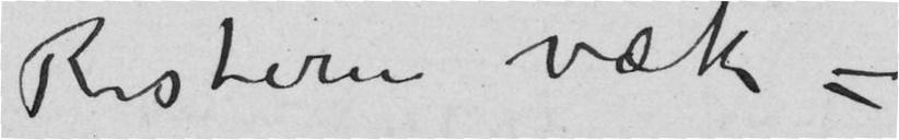Resterne væk –
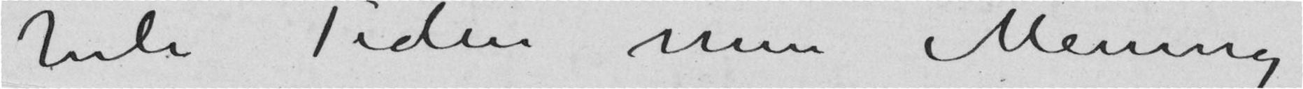hele Tiden min Mening
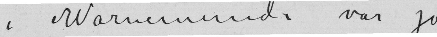i Warnemünde var jo
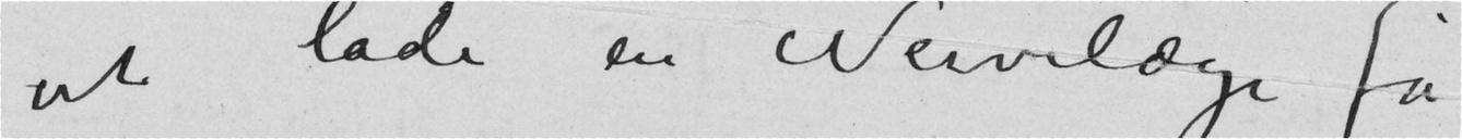at lade en Nervelæge få
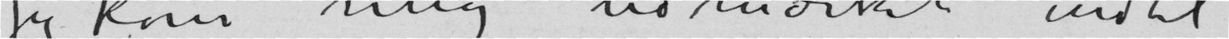jeg kom mig udmærket indtil
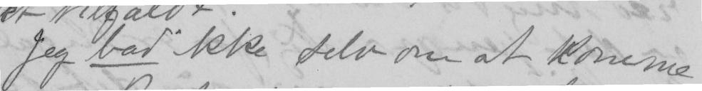Jeg bad ikke selv om at komme
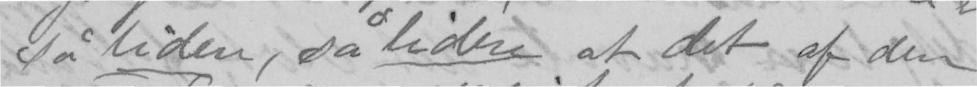så liden, så liden at det af den
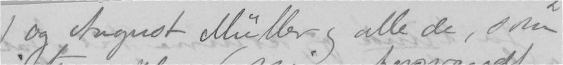og August Müller og alle de, som
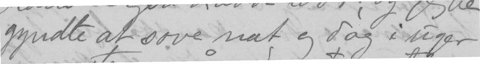gyndte at sove nat og dag i uger
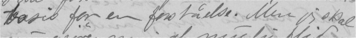basis for en forståelse. Men jeg skal
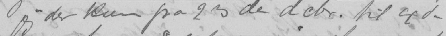jeg der kun fra 23 de dcbr. til 4de
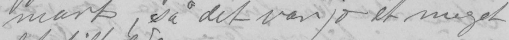marts, så det var jo et meget
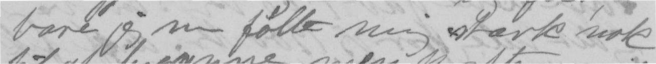bare jeg nu følte mig stærk nok
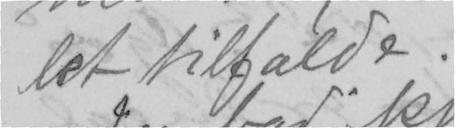let tilflde.
